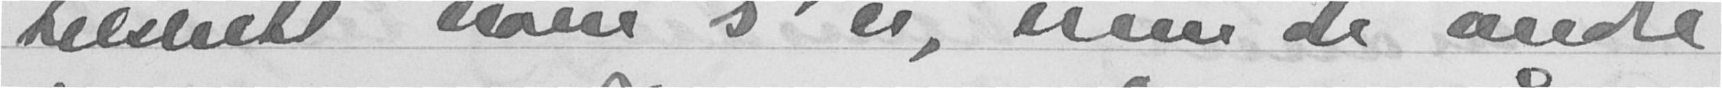tilslutt noen s'er, men de andre
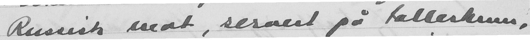Russisk mat, servert på tallerken,
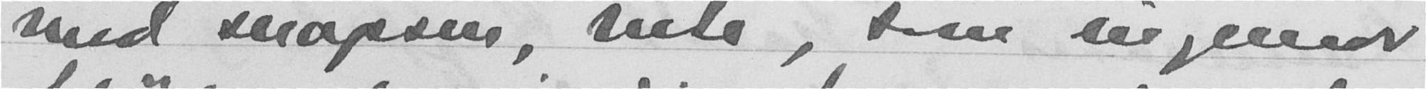med snapsen, inte, som ingeniør
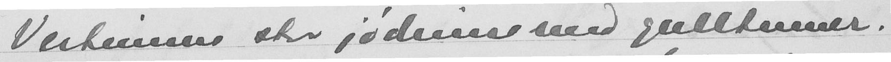Vertinnen stor jødinne med gulltenner.
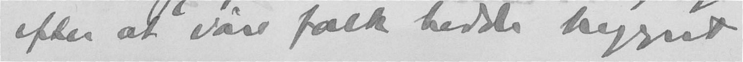efter at våre folk hadde begynt.
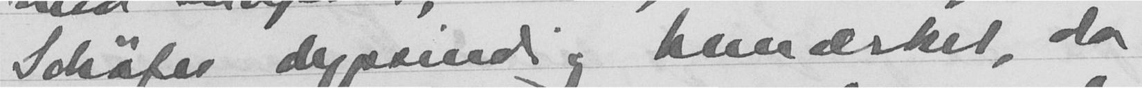Schäfer dypsindig bemærket, da
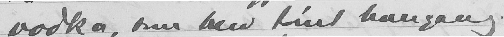vodka, som blev tømt hvergang
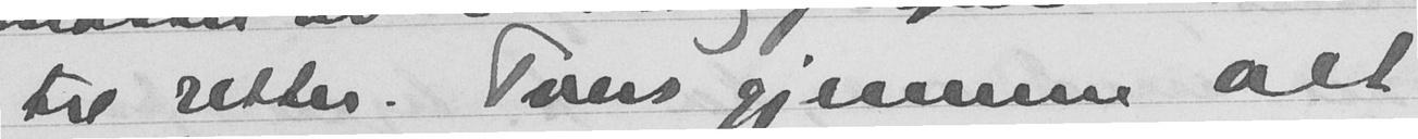tre retter. Tvers gjennom alt
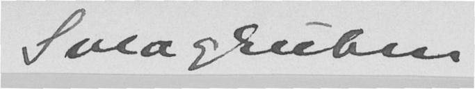Sveagruben
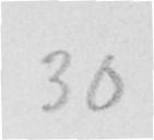30
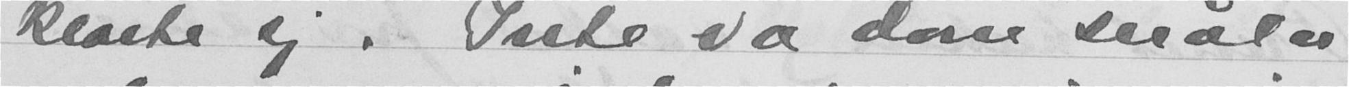klarte sig. Inte va dom snåler
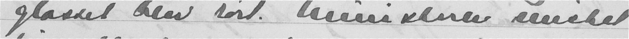glasset blir rørt. Ministeren smitet
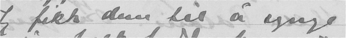Jeg fikk dem til å synge
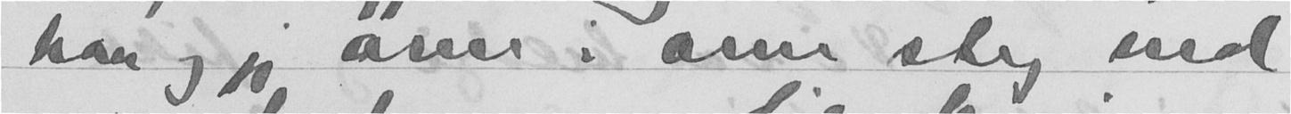han og jeg i arm i arm steg ned
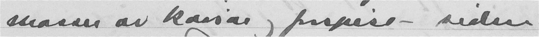masser av kaviar og forspise - siden
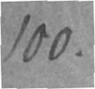100.
